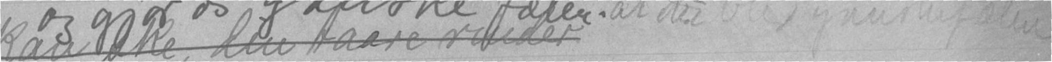og gjør os ganske fælen.
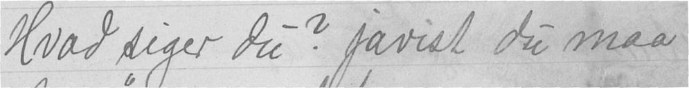Hvad siger du? Javist du maa
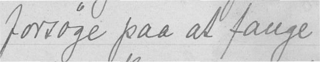forsøge paa at fange
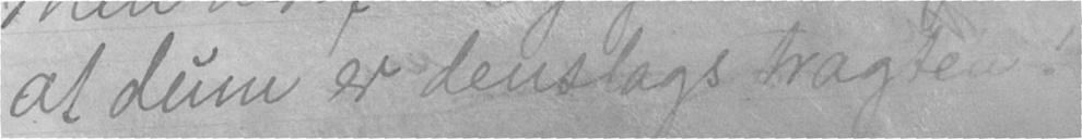at dum er denslags tragten!
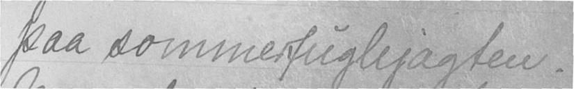paa sommerfuglejagten.
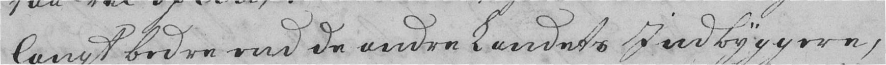langt bedre end de andre Landets Indbyggere,
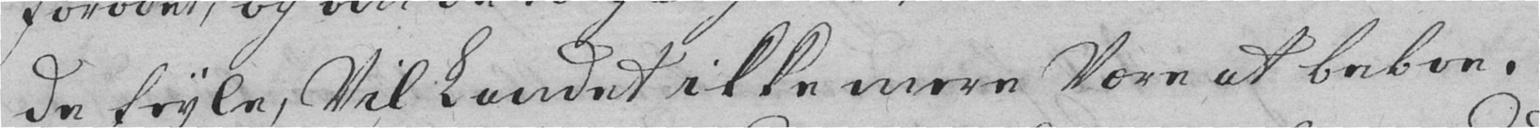de feyle, vil Landet ikke mere Være at beboe.
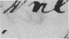Vel
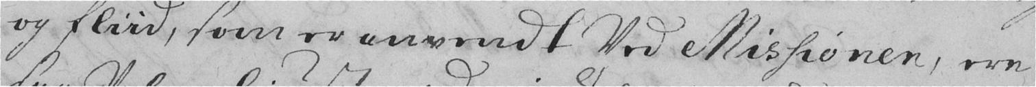og fliid, som er anvendt Ved Missionen, ere
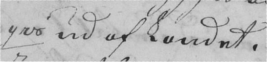gis ud af Landet.
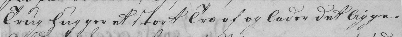Trug hugger et stort Træ af og lader det ligge.
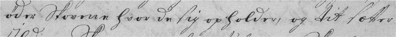øder Skovene hvor de sig opholder, og tit sætter
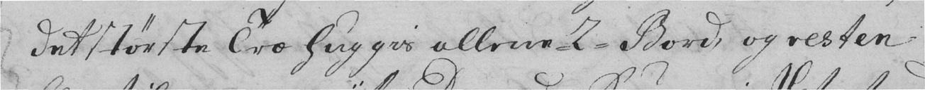det største Træ huggis allene 2 Bord, og resten
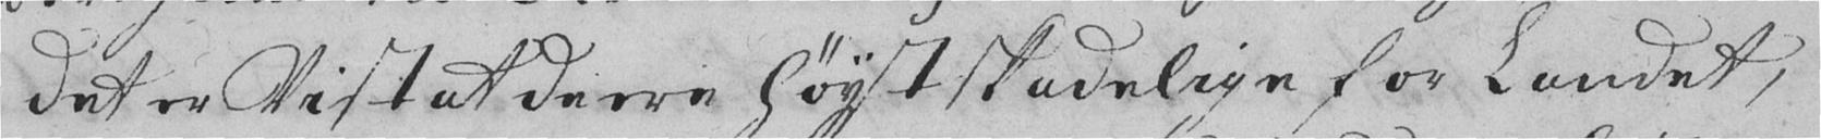det er Vist at de ere høyst skadelige for Landet,
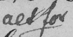alt for
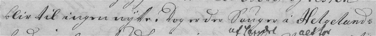blir til ingen nytte. Dog er der Sauger i Helgelands
